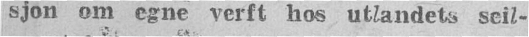sjon om egne verft hos utlandets seil-
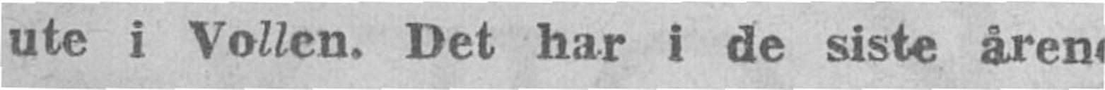ute i Vollen. Det har i de siste årene
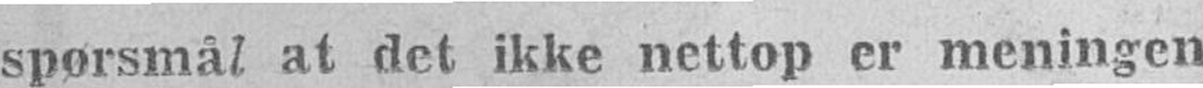spørsmål at det ikke nettop er meningen
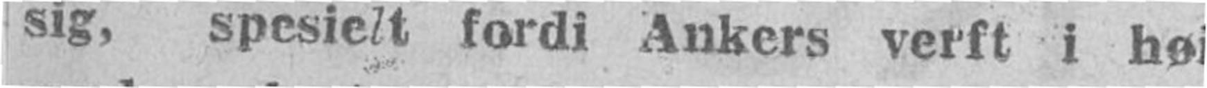sig, spesielt fordi Ankers verft i høi
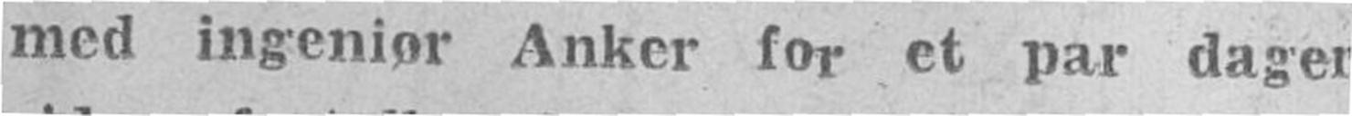med ingeniør Anker for et par dager
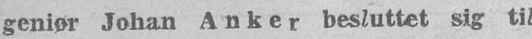geniør Johan Anker besluttet sig til
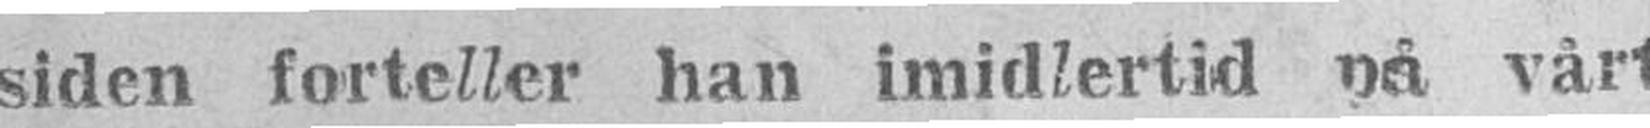siden forteller han imidlertid på vårt
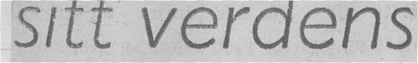sitt verdens
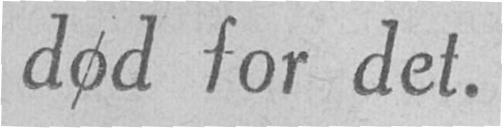død for det.
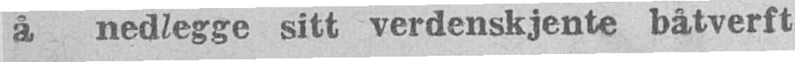å nedlegge sitt verdenskjente båtverft
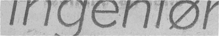Ingeniør
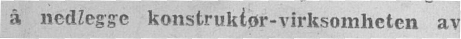å nedlegge konstruktør-virksomheten av
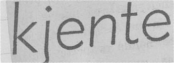kjente
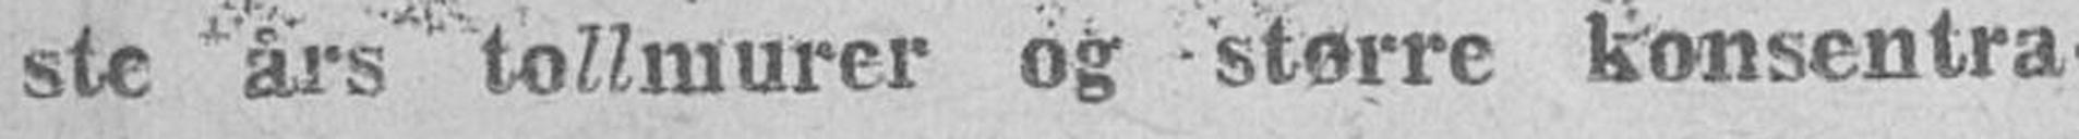ste års tollmurer og større konsentra-
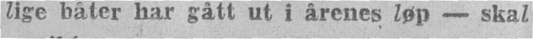lige båter har gått ut i årenes løp — skal
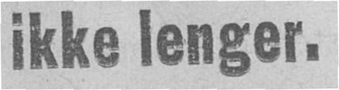ikke lenger.
